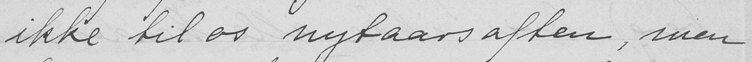ikke til os nytaarsaften, men
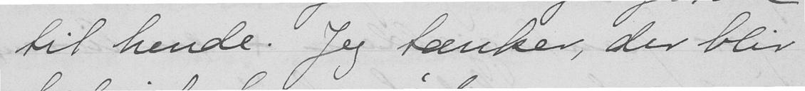til hende. Jeg tænker, der blir
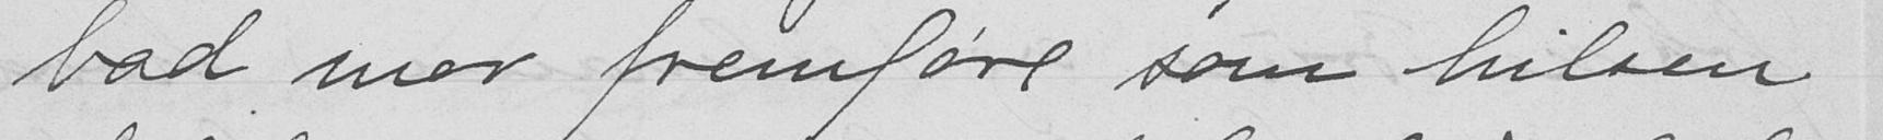bad mor fremføre som hilsen
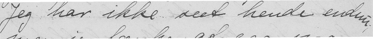Jeg har ikke seet hende endnu,
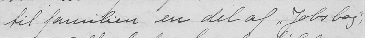til familien en del af "Jobs bog",
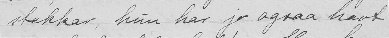stakkar, hun har jo ogsaa havt
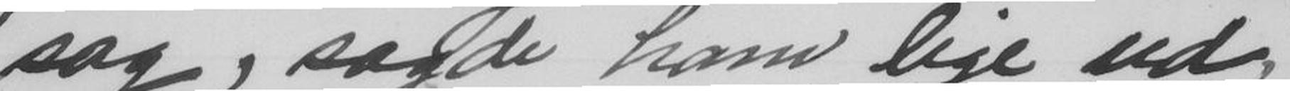sag, sagde ham lige ud,
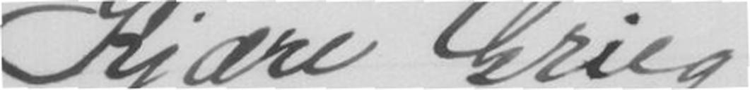Kjære Grieg!
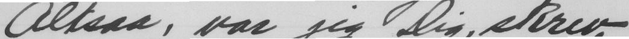Altsaa, var jeg Dig, skrev
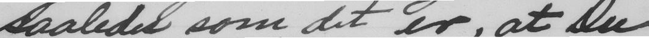saaledes som det er, at Du
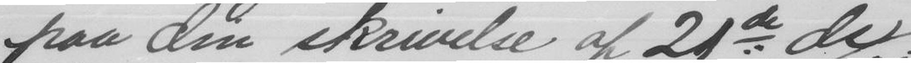paa din skrivelse af 21de dc/;
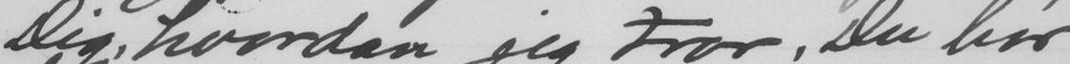Dig, hvordan jeg tror, Du bør
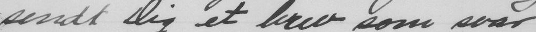sendt Dig et brev som svar
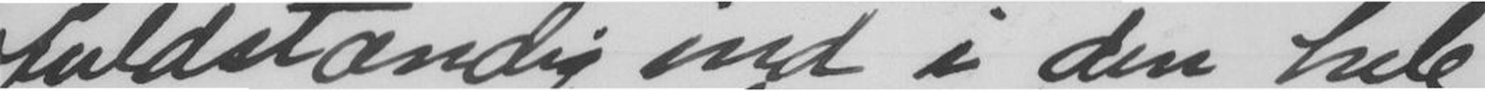fuldstændig ind i den hele
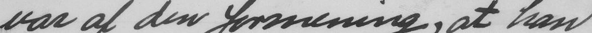var af den formening, at han
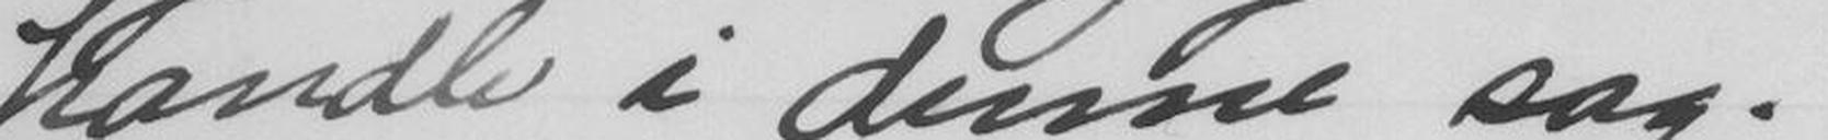handle i denne sag.
